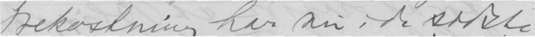Bekostning har nu i de sidste
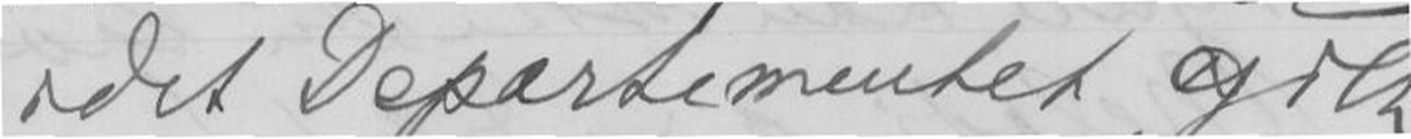idet Departementet gik
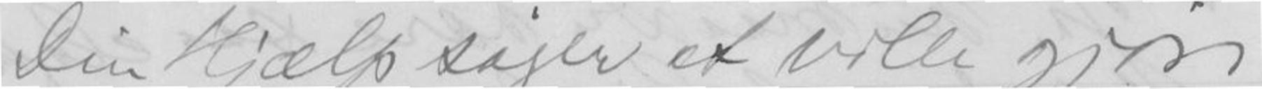Din Hjælp søger at ville gjøre
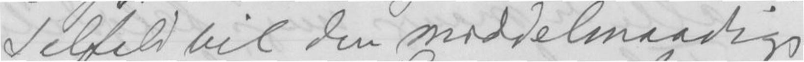Ialfald vil den middelmaadige
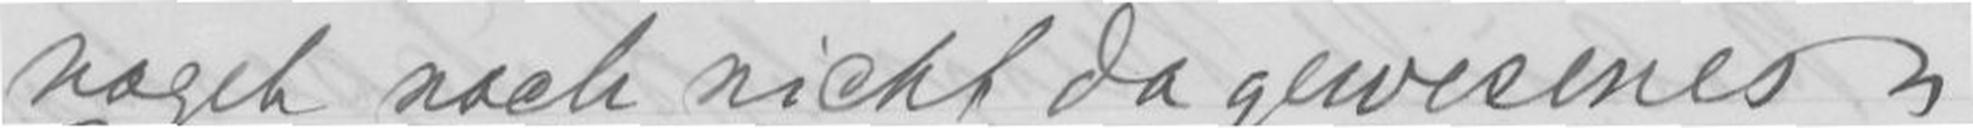noget nach nicht da gewesenes.
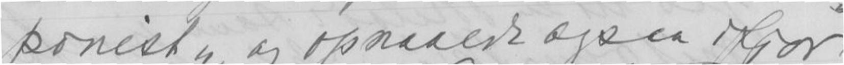ponist", og opnaaede ogsaa ifjor
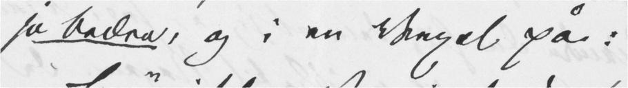jo bedre, og i en Vexel på:
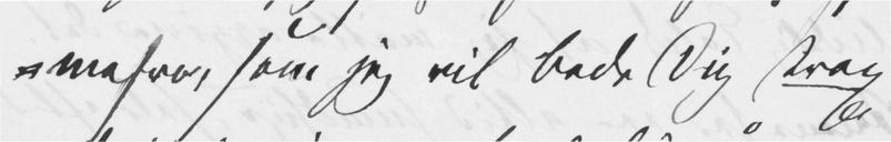mefra, som jeg vil bede Dig strax
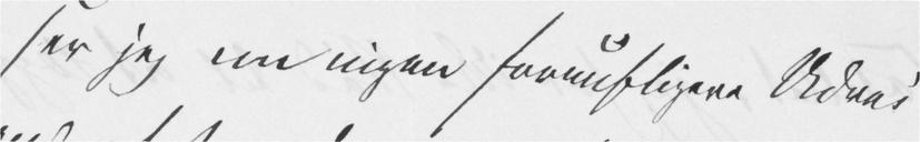ser jeg nu ingen fornuftigere Udvei
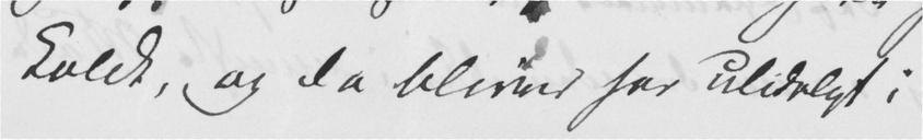koldt, og da bliver her ulideligt i
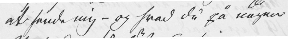at sende mig – og hvad Du på nogen
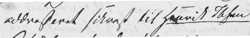addresseret sikrest til Henrik Ibsen
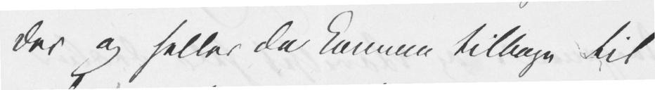der og heller da komme tilbage til
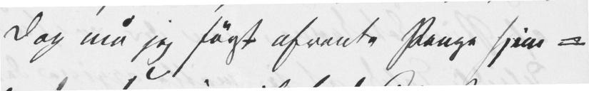Dog må jeg først afvente Penge hjem-
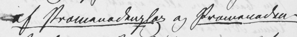af Promenadenplaz og Promenaden-
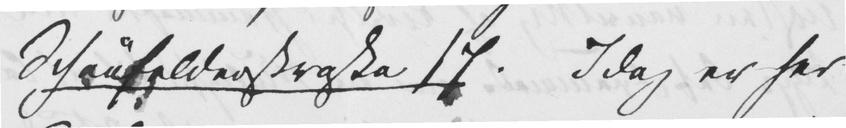Schönfeldenstrasse 17. Idag er her
